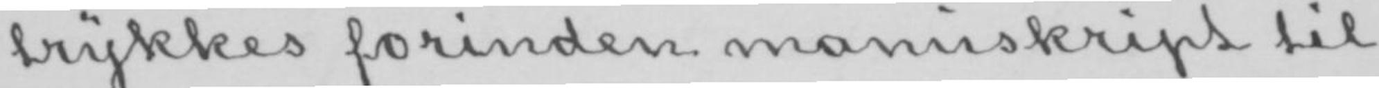trykkes forinden manuskript til
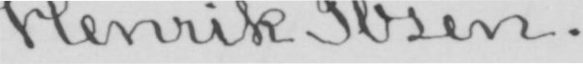Henrik Ibsen.
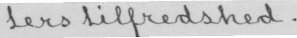ters tilfredshed.
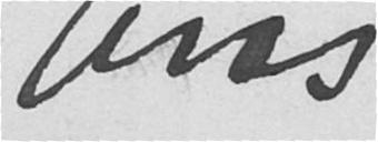Jens
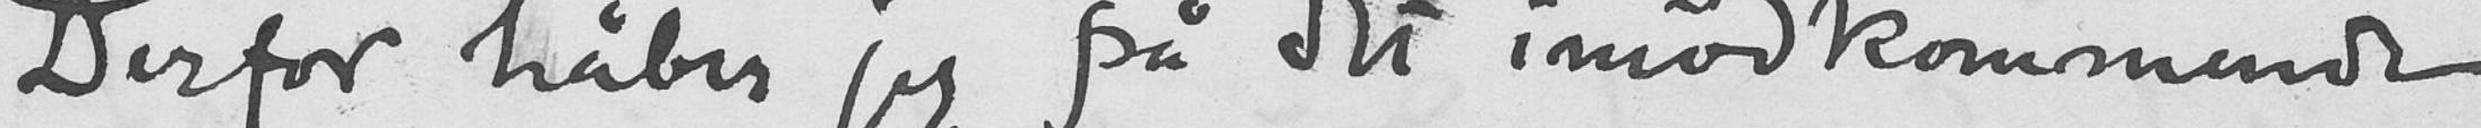Derfor håber jeg på det imødkommende
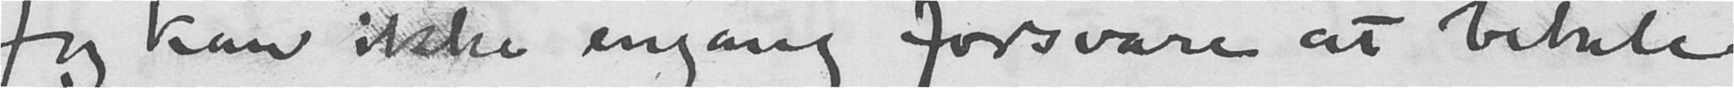Jeg kan ikke engang forsvare at betale
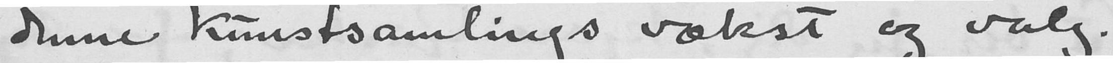denne kunstsamlings vækst og valg.
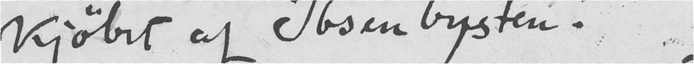kjøbet af Ibsenbysten!
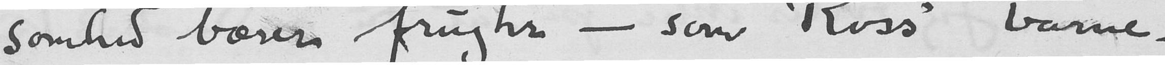somhed bærer frugter - som Ross' "barne-
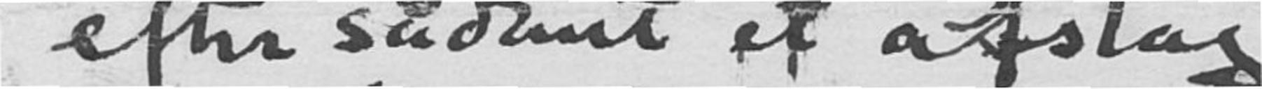efter sådantet afslag
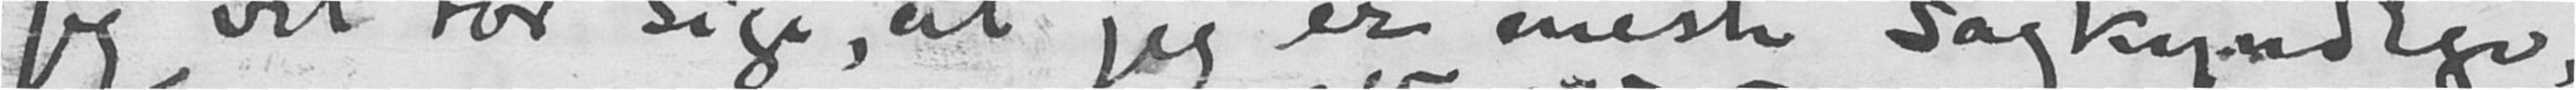jeg vel tør sige, at jeg er eneste sagkyndige,
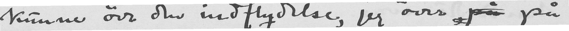kunne øve den indflydelse, jeg øver på på
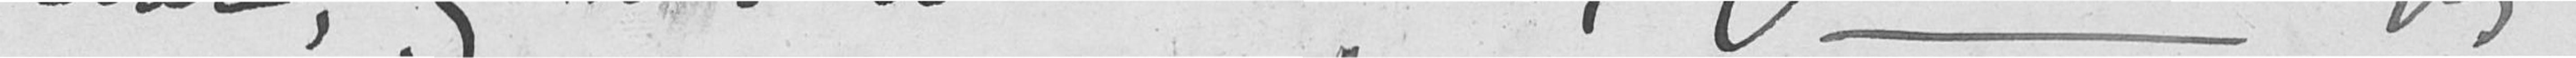svar, og når det vi har det, garanterer jeg
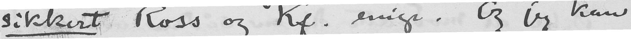sikkert Ross og Kf. enige. Og jeg kan
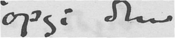opgi den
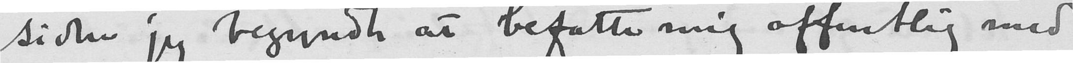siden jeg begyndte at befatte mig offentlig med
